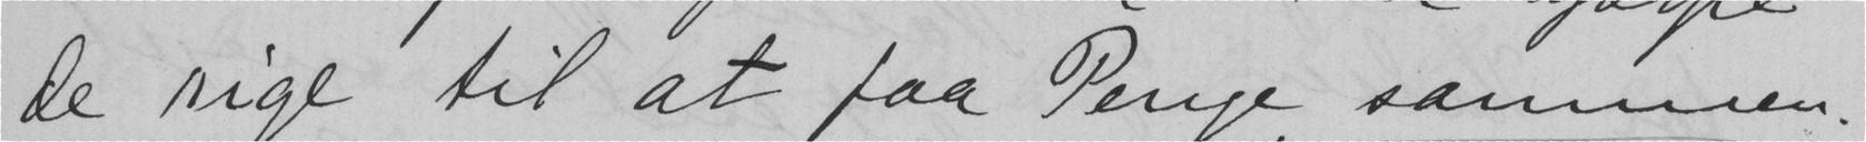de rige til at faa Penge sammen.
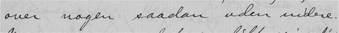over nogen saadan uden videre.
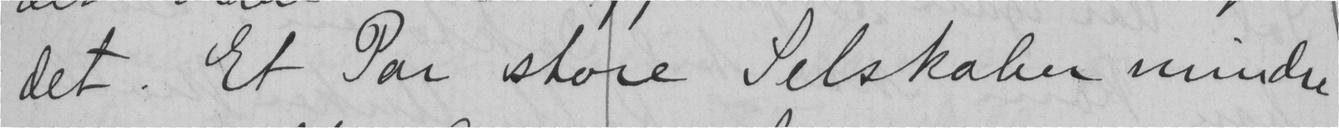det. Et Par store Selskaber mindre
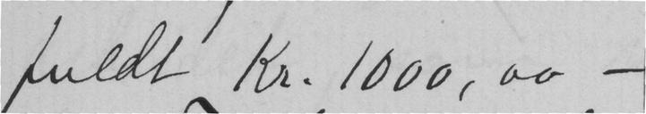fuldt Kr. 1000, 00 -
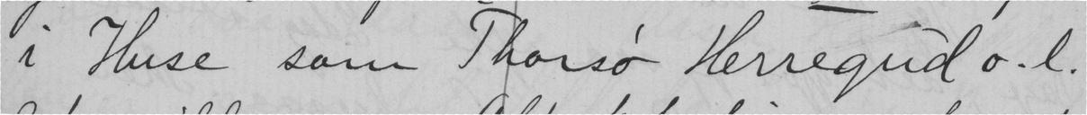i Huse som Thorsø Herregud o.l.
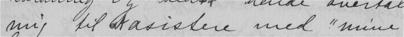mig til at asistere med "mine
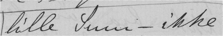lille Sum - ikke
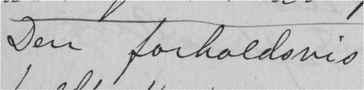Den forholdsvis
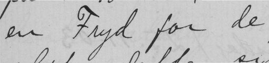en Fryd for de
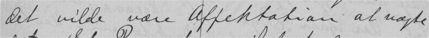det vilde være affektation at nægte
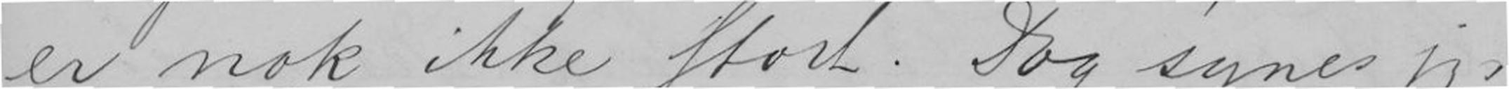er nok ikke stort. Dog synes jeg
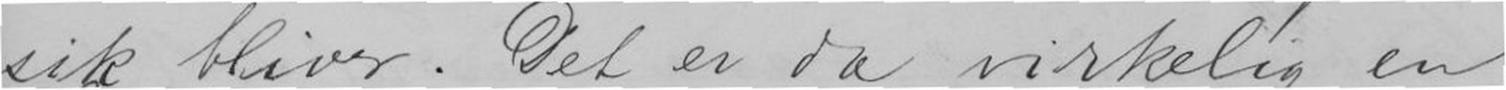sik bliver. Det er da virkelig en
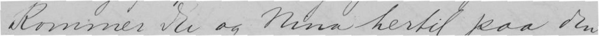Kommer Du og Nina hertil paa den
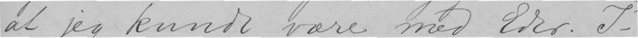at jeg kunde være med Eder. I-
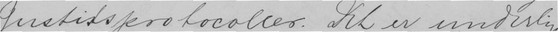Justitsprotocoller. Det er underligt
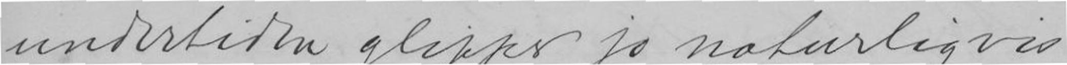undertiden glipper jo naturligvis
